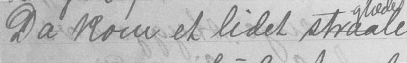Da kom et lidet straale
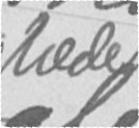glæde
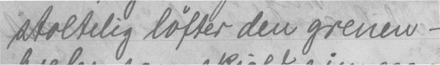stoltelig løfter den grenen -
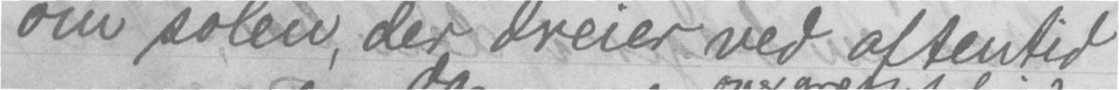om solen, der dreier ved aftentid
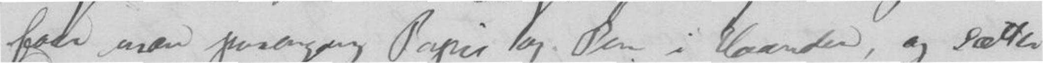faar man paaengang Papir og Pen i Haanden, og sætter
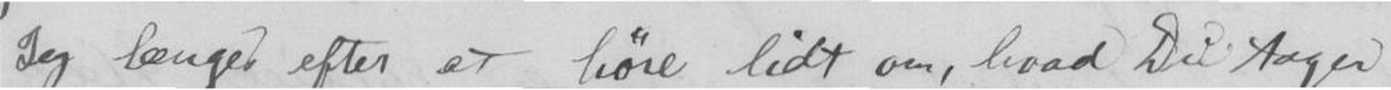Jeg længes efter at høre lidt om, hvad Du tager
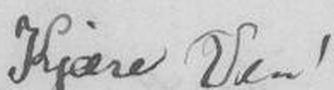Kjære Ven!
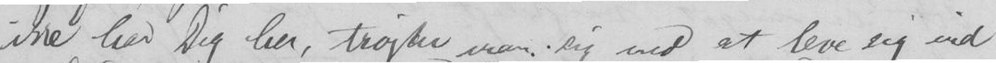ikke har Dig her, trøste man sig ved at leve sig ind
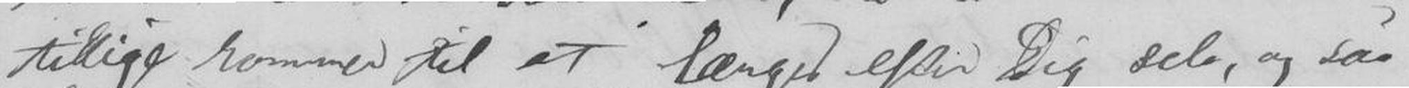tillige kommer til at længes efter Dig selv, og saa
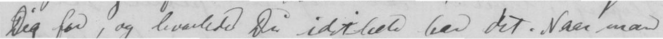Dig for, og hvorledes du idethele har det. Naar man
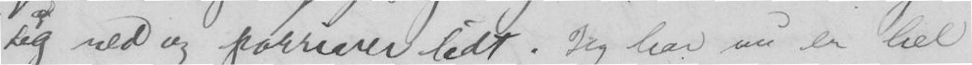sig ned og passiarer lidt. Jeg har nu en hel
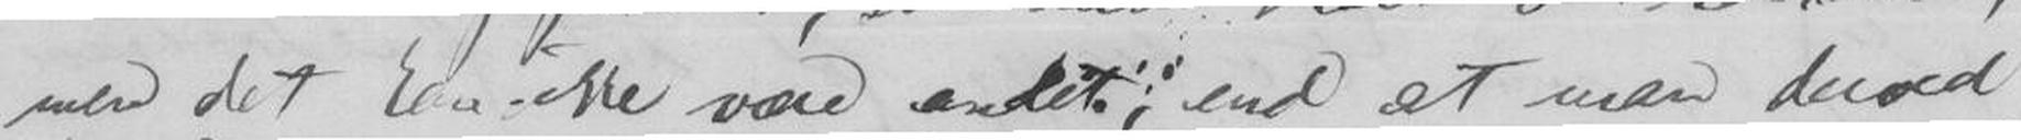men det kan ikke være andet; end at man derved
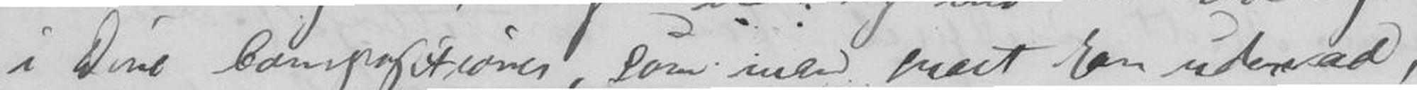i Dine Compositioner, som men mest kan udenad,
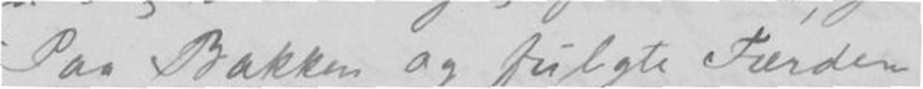Paa Bakken og fulgte Færden,
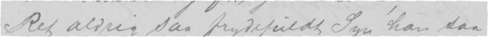Ret aldrig saa frydefuldt Syn han saa
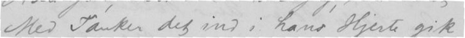Med Tanken det ind i hans Hjerte gik;
